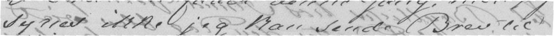synes ikke jeg kan sende Brev til
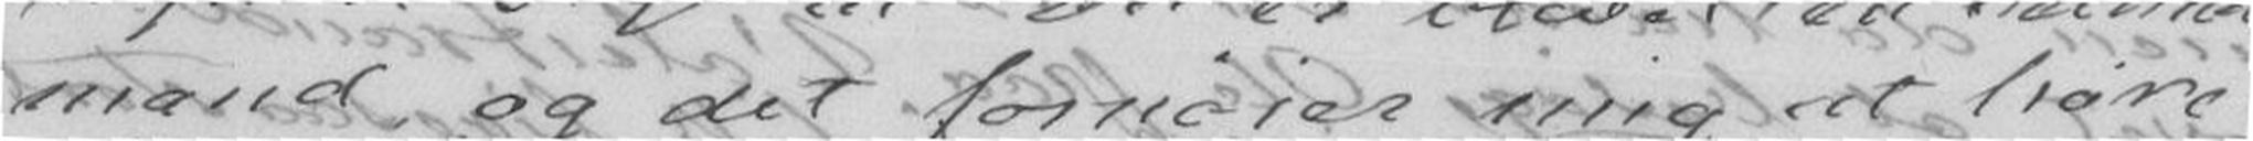mand, og det fornøier mig at høre.
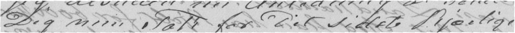Dig min Tak for Dit sidste kjærlige
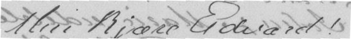Min kjære Edvard!
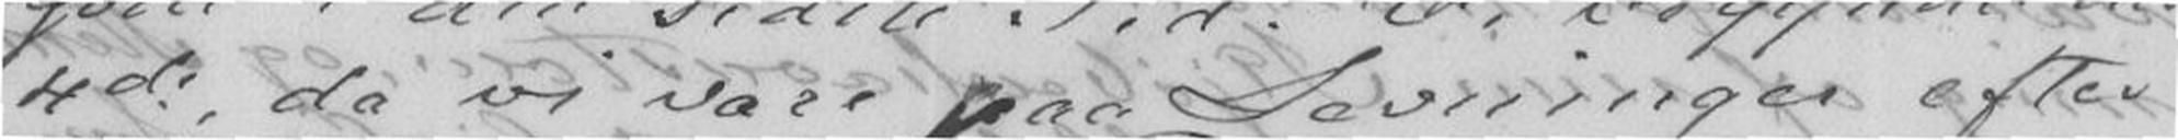4de, da vi vare paa Levninger efter
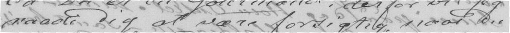raade Dig at være forsigtig naar Du
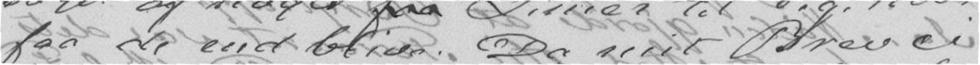faa de end bliver. Da mit Brev ei
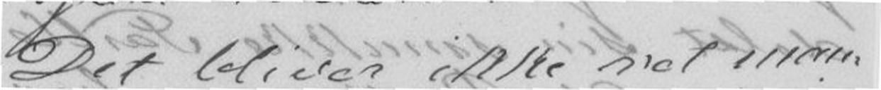Det bliver ikke ret man-
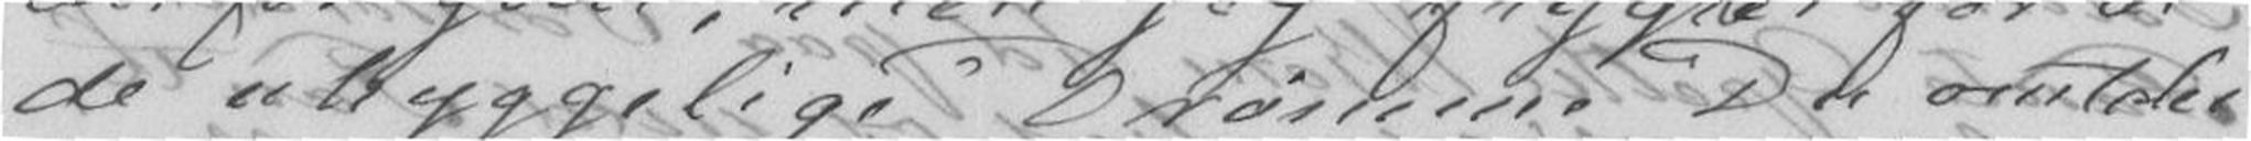de uhyggelige Drømme Du omtaler
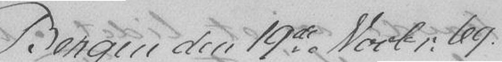Bergen den 19de Novbr. 69.
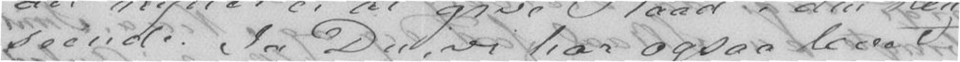seende. Ja Du, vi har ogsaa levet
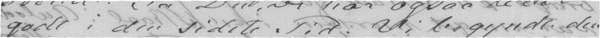godt i den sidste Tid. Vi begyndte den
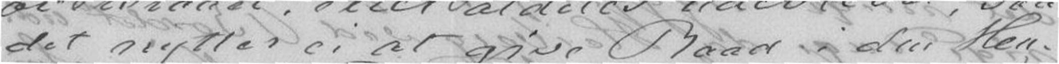det nytter ei at give Raad i den Hen-
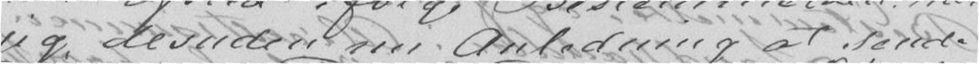jeg desuden nu Anledning at sende
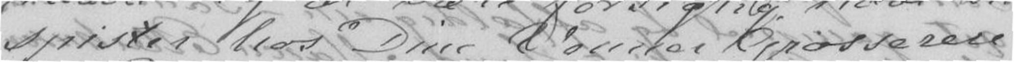spiser hos Dine Venner Grossere
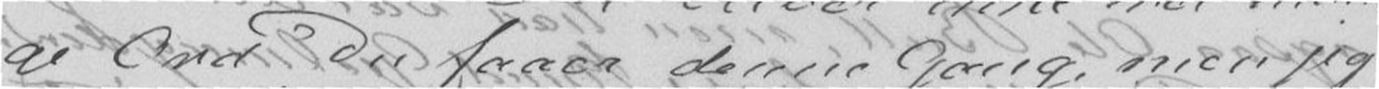ge Ord du faaer denne Gang, men jeg
